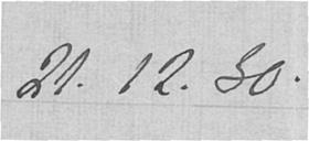21.12.30.
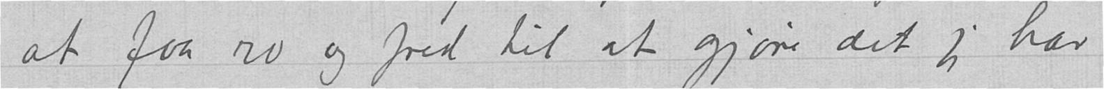at faa ro og fred til at gjøre det jeg har
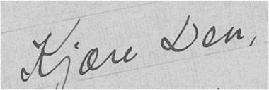Kjære Dea,
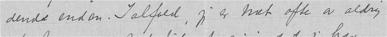dende enden. Ialfald, jeg er træt ofte av aldrig
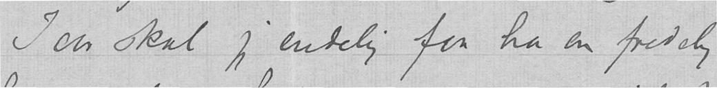I aar skal jeg endelig faa ha en fredelig
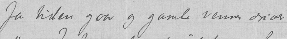Ja tiden gaar og gamle venner driver
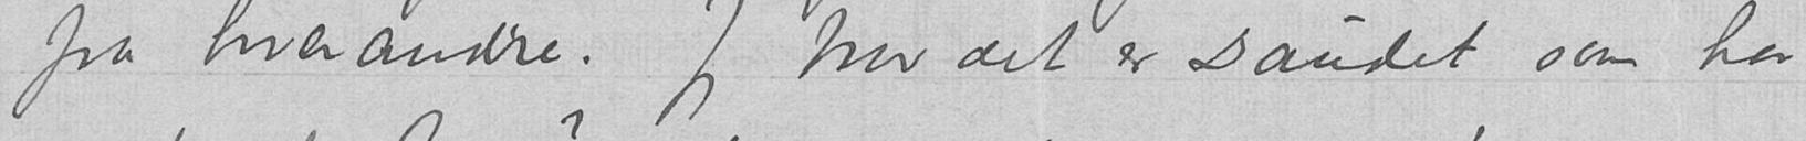fra hverandre. Jeg tror det er Daudet som har
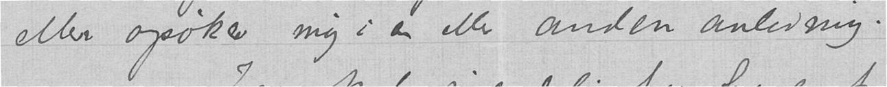eller opsøker mig i en eller anden anledning.
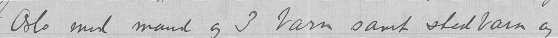Oslo med mand og 3 barn samt stedbarn og
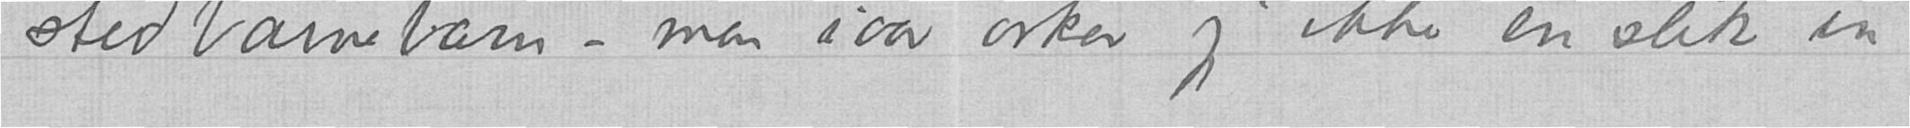stedbarnebarn - men iaar orker jeg ikke en slik in-
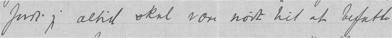fordi jeg altid skal være nødt til at befatte
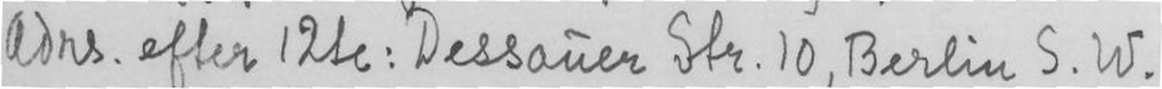Adrs. efter 12te: Dessauer Str.10, Berlin S.W.
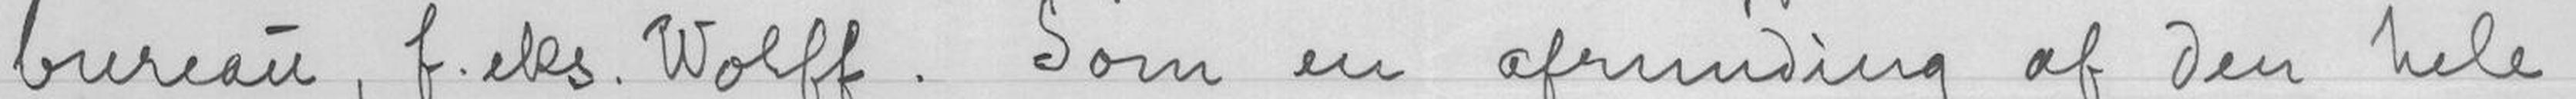bureau, f. eks. Wolff. Som en afrunding af den hele
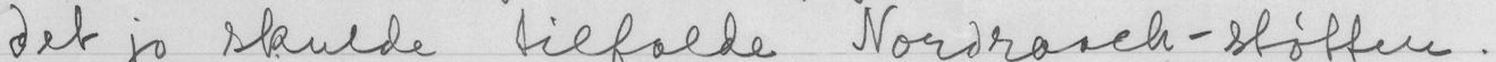det jo skulde tilfalde Nordraach-støtten.
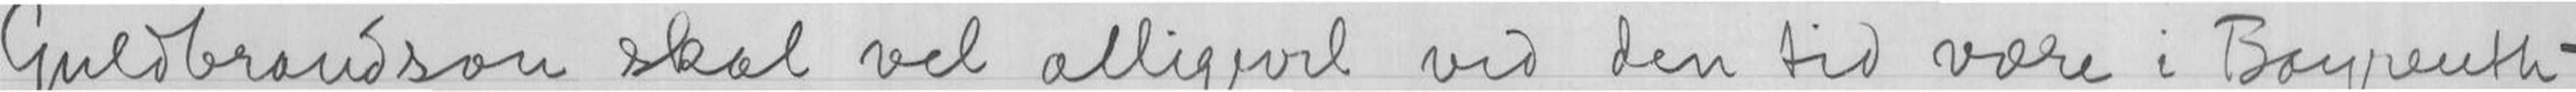Guldbrandson skal vel alligevel ved den tid være i Bayreuth -
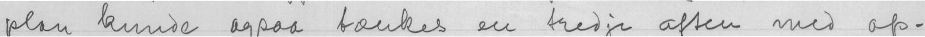plan kunde ogsaa tænkes en tredje aften med op-
